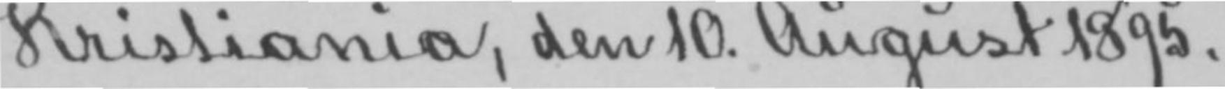Kristiania, den 10. August 1895.
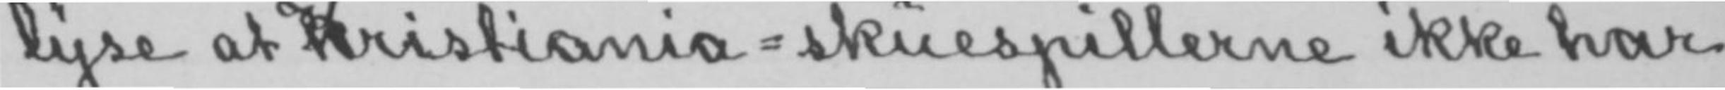lyse at kKristiania-skuespillerne ikke har
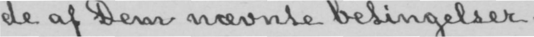de af Dem nævnte betingelser.
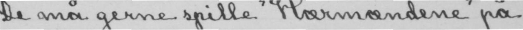De må gerne spille «Hærmændene» på
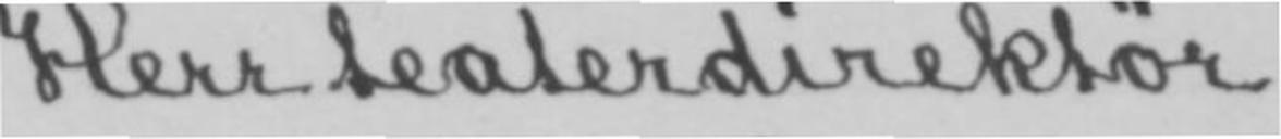Herr teaterdirektør
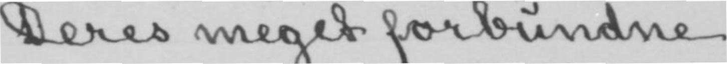Deres meget forbundne
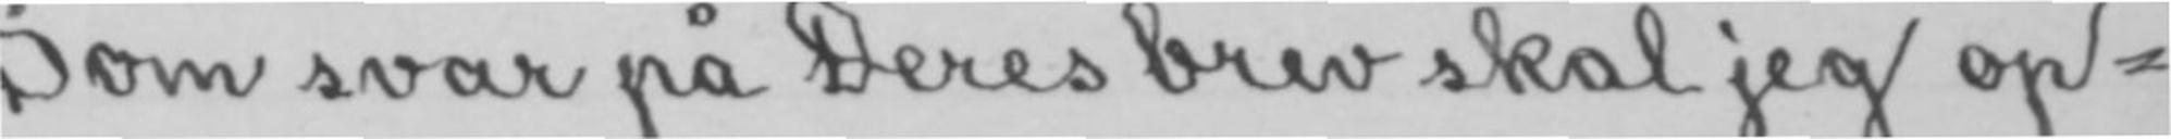Som svar på Deres brev skal jeg op-
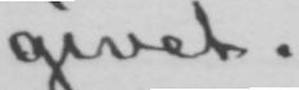givet.
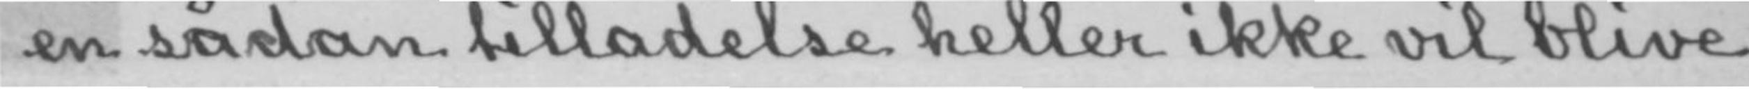en sådan tilladelse heller ikke vil blive
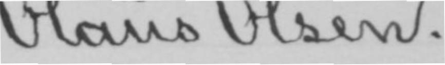Olaus Olsen.
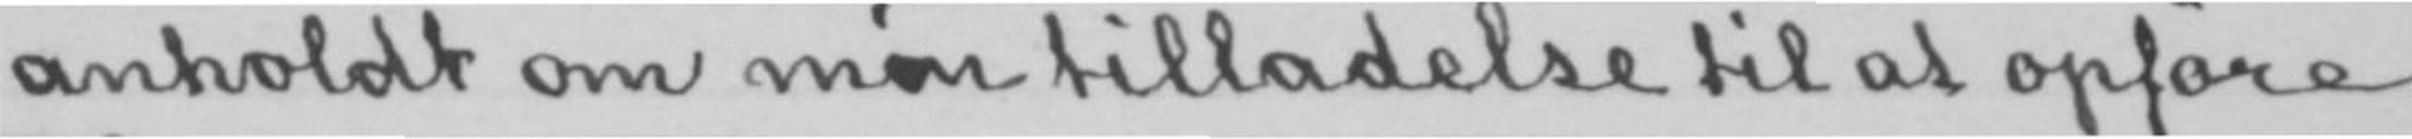anholdt om min tilladelse til at opføre
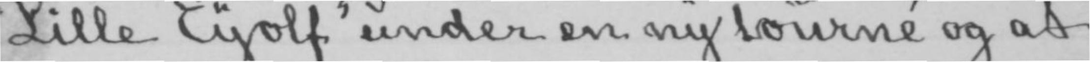«Lille Eyolf» under en ny tourné og at
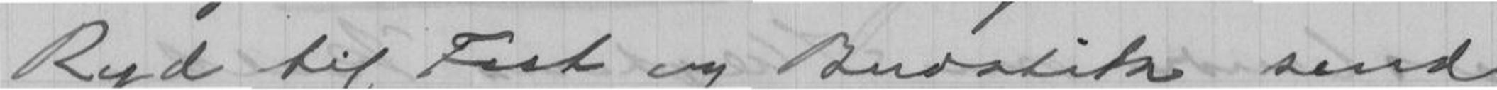Ryd til Fest og Budstik send
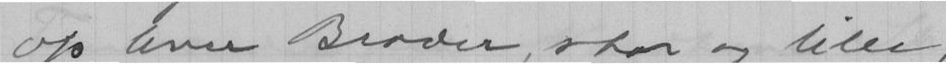op hver Broder, stor og liden,
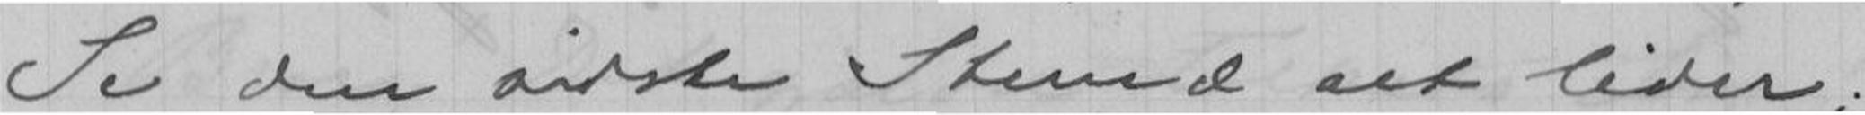Se den sidste Stund alt lider;
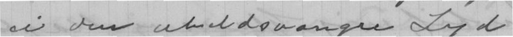ei den uheldsvangre Lyd?
